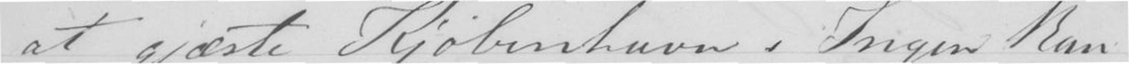at gjæste Kjøbenhavn, Ingen kan
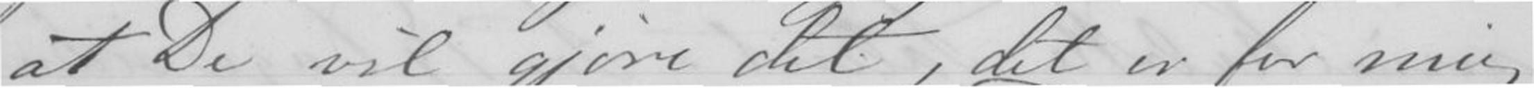at De vil gjøre det, det er for mig
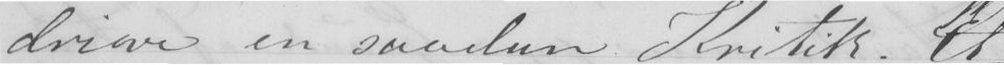drive en saadan Kritik. Et
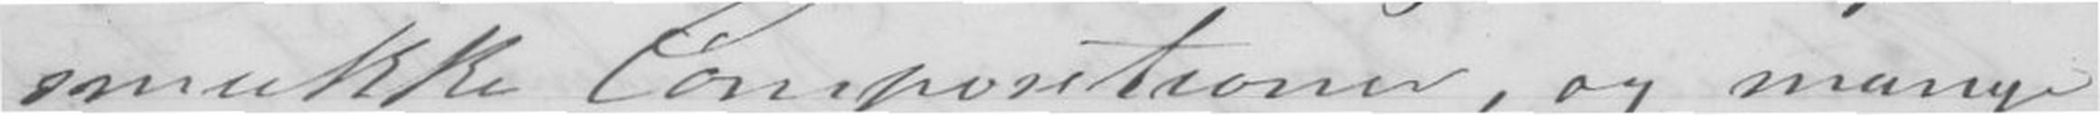smukke Compositioner, og mange
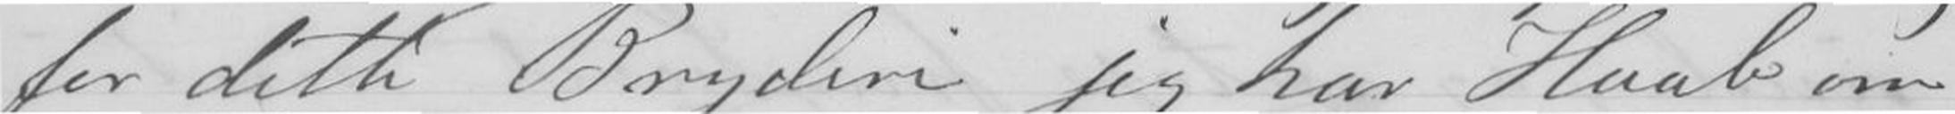for dette Bryderi jeg har Haab om
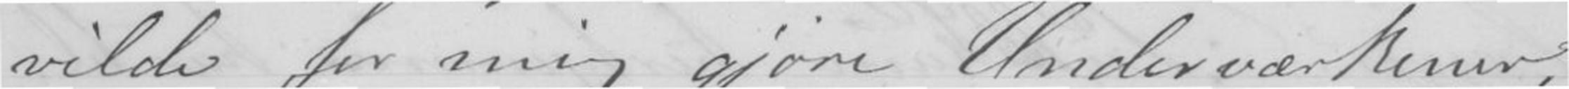vilde for mig gjøre Underværkener
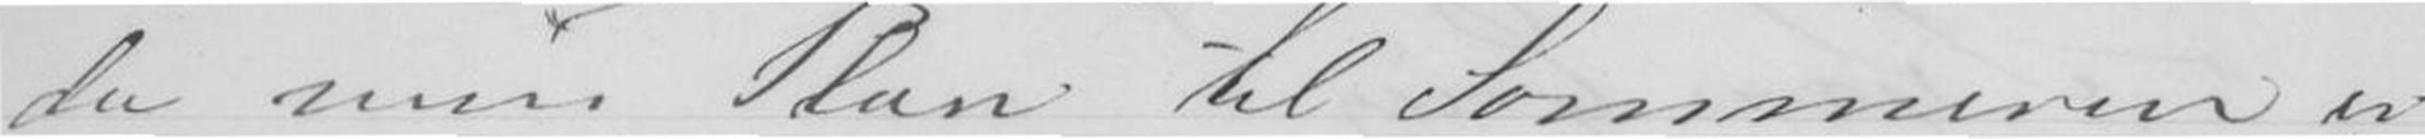da min Plan til Sommeren er
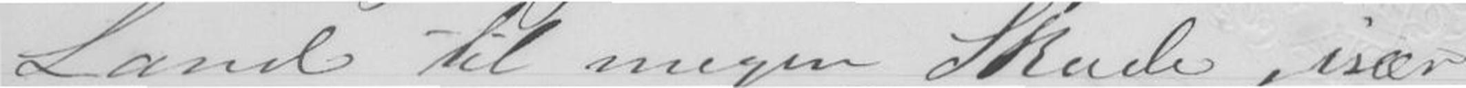Land til megen Skade, især
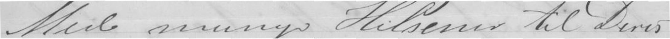Med mange Hilsener til Deres
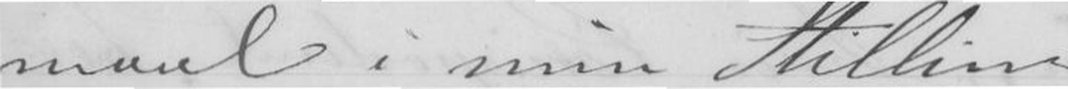maal i min Stilling.
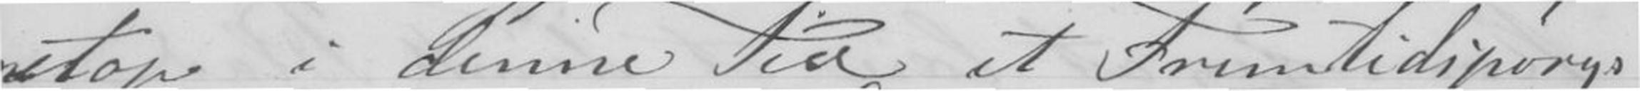netop i denne Tid et Fremtidspørgs-
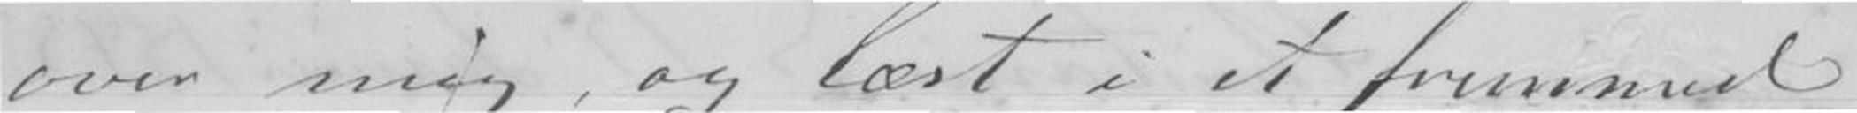over mig, og læst i et fremmed
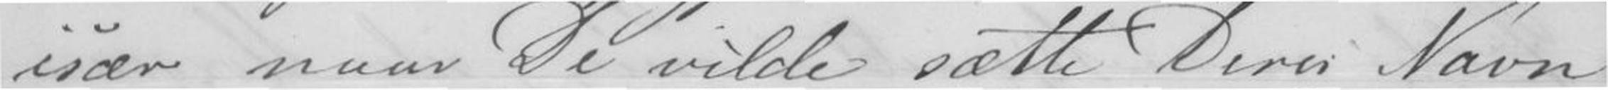især naar De vilde sætte Deres Navn
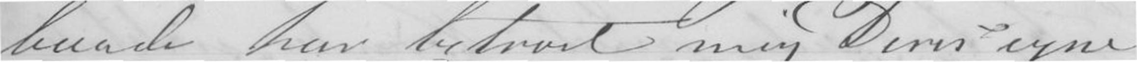baade har betroet mig Deres egne
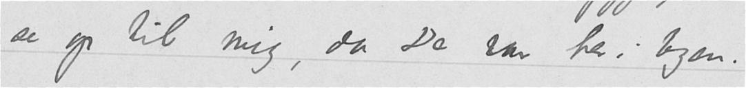se op til mig, da De var her i byen.
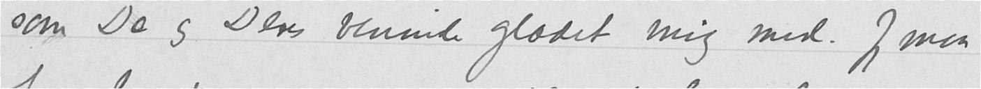som De og Ders veninde glædet mig med. Jeg maa
